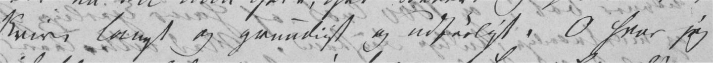skrive langt og grundigt og udførligt. O hvor jeg
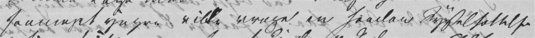saameget yngre vilde vrage en saadan Sysselsættelse
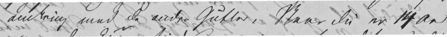omkring med de andre Gutter, Naar Du er 14 Aar
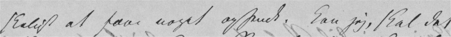skeligt at faae noget opsendt, kan jeg, skal det
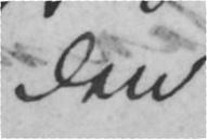den
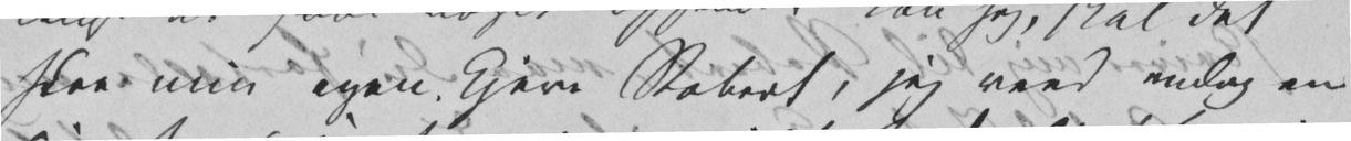skee min egen, kjere Robert, jeg veed endog en
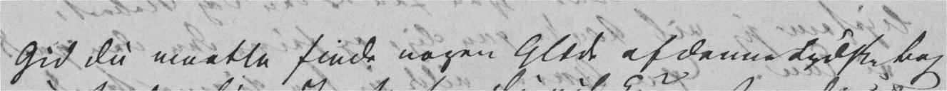Gid Du maatte finde nogen Glæde af denne tydske Bog
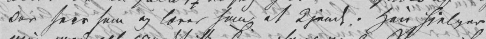der seer ham og lærer ham at kjende. Han hjelper
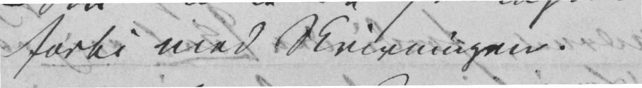forbi med Skrivningen.
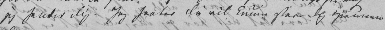jeg sender Dig. Jeg haaber Du vil kunne stave Dig igjennem
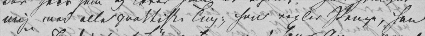mig med alle praktiske Ting: han vexler Penge, han
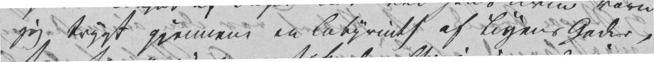jeg trygt gjennem en Labyrinth af Byens Gader,
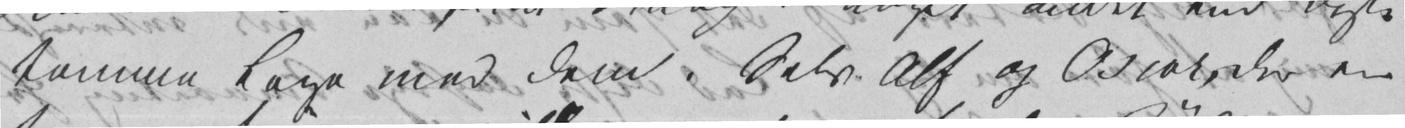tomme Lege med dem. Selv Alf og Oscar, der ere
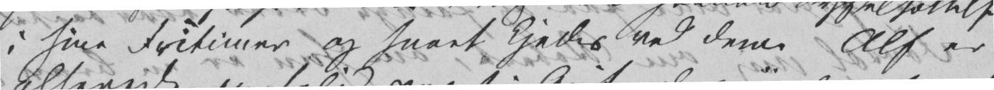i sine Fritimer og snart kjedes ved dem. Alf er
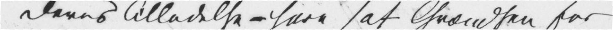Deres Tilladelse – have sat Grændsen for
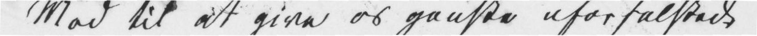Mod til at give os ganske uforfalskede
